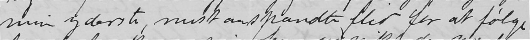min yderste, mest anspændte flid for at følge
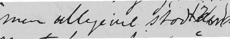men alligevel stod jeg der
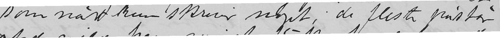som når hun skriver noget; de fleste påstår
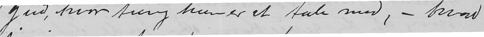gud, hvor tung hun er at tale med, - hvad
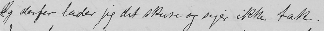Og derfor lader jeg det skure og siger ikke tak.
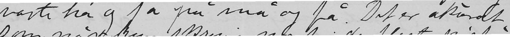svarte ha og ja på må og få. Det er akurat
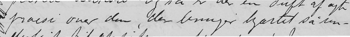poesi over den, der bringer hjærtet så un-
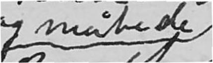måbende
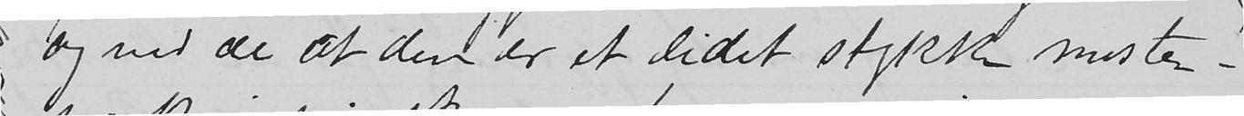og ved de at den er et lidet stykke mester-
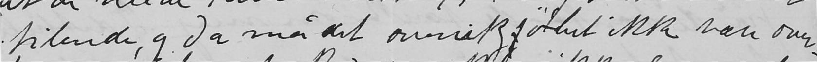tilende, og da må det ovenikjøbet ikke være over-
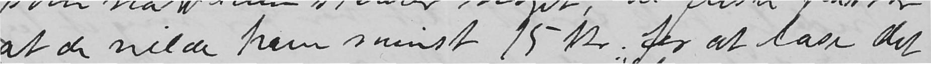at de vilde have minst 15 kr. for at læse det
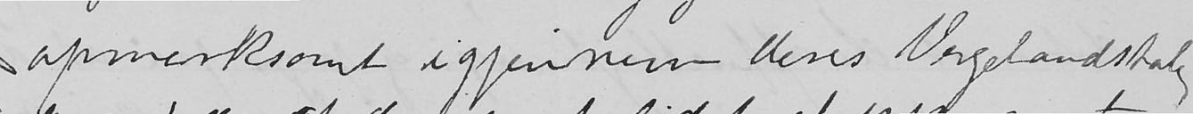opmerksomt igjennem deres Vergelandstale
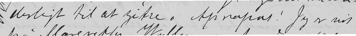derligt til at zitre. Apropos! Jeg er vis
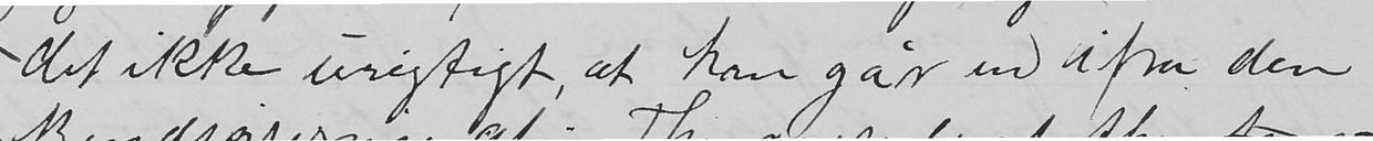det ikke urigtigt, at han går ud ifra den
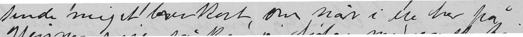sende mig et brevkort, om når i ere her på
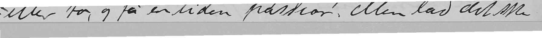eller to, og få en liden passiar. Men lad det ske
